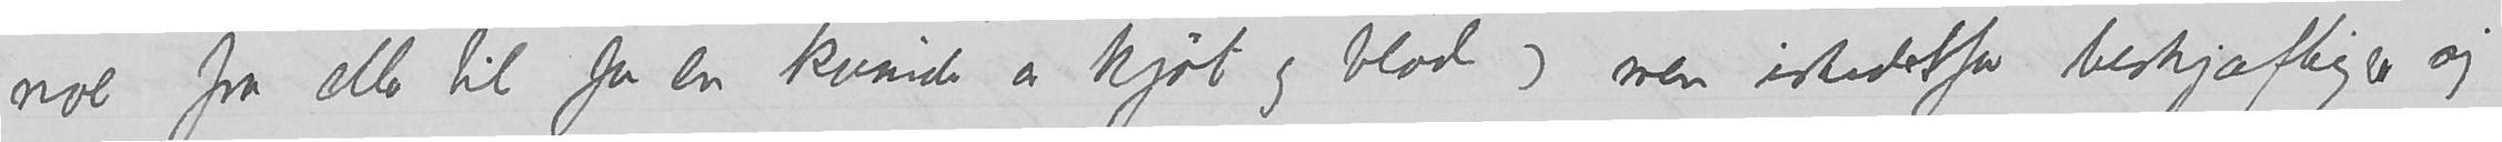noe fra eller til for en kvinde av kjøt og blod) men istedetfor beskjæftiger sig
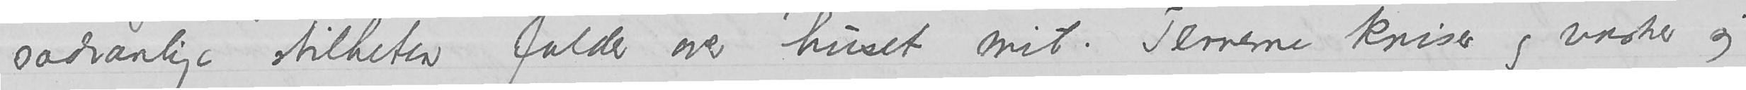sædvanlige stilheten falder over huset mit.  Ternerne kniser og vasker sig
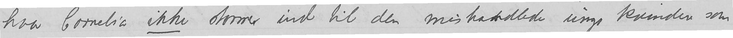hvor Cornelia ikke stormer ind til den mishandlede unge kvinden som
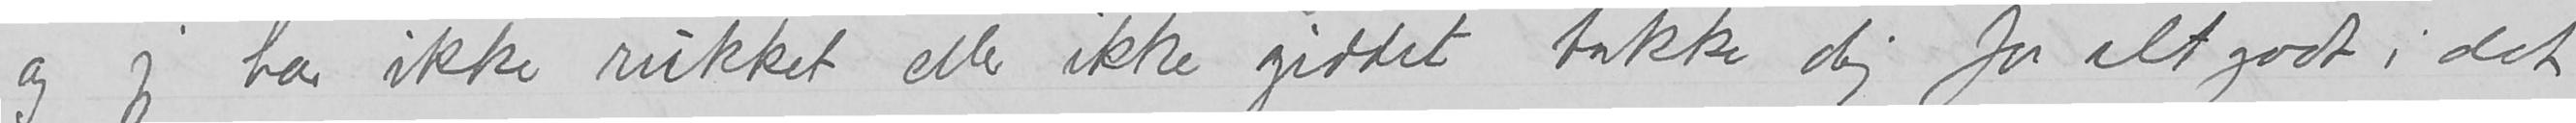og jeg har ikke rukket eller ikke giddet takke dig for alt godt i det
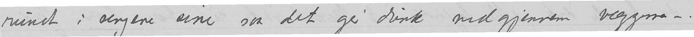rundt i sengene sine saa det gir dunk ned gjennem veggerne –.
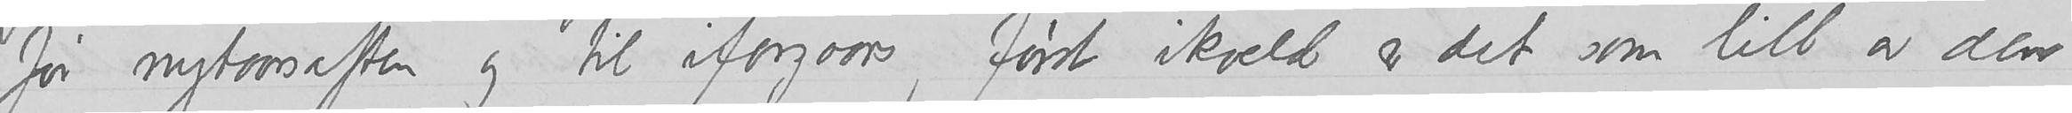før nytaarsaften og til iforgaars, først ikveld er det som litt av den
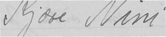Kjære Nini,
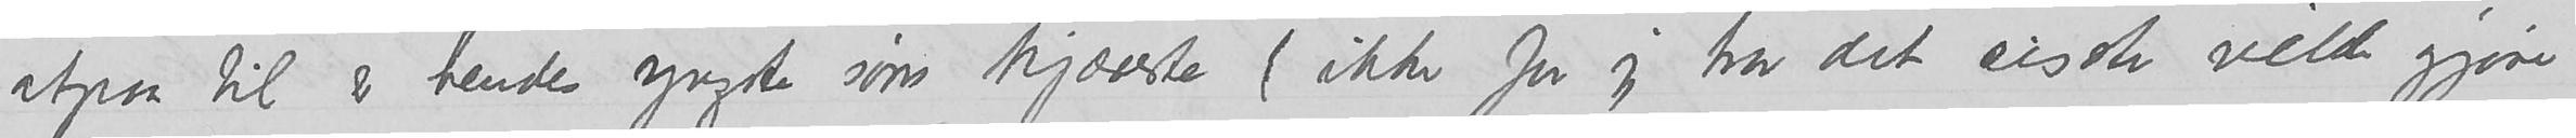atpaa til er hendes yngste søns kjæreste (ikke for jeg tror det sisste vilde gjøre
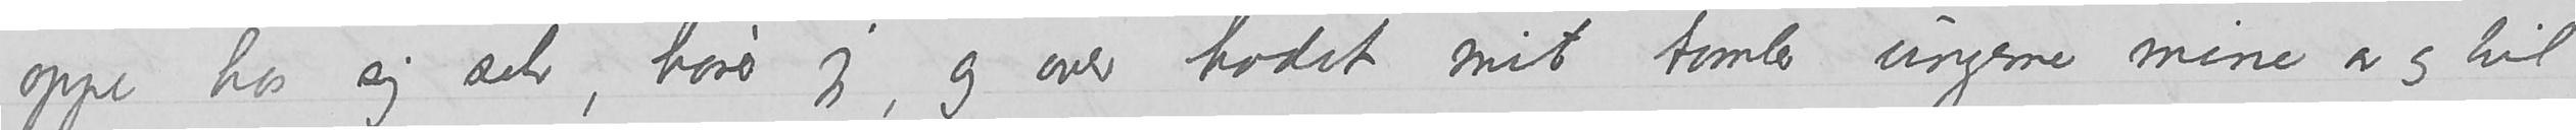oppe hos sig selv, hører jeg, og over hodet mit tomler ungerne mine av og til
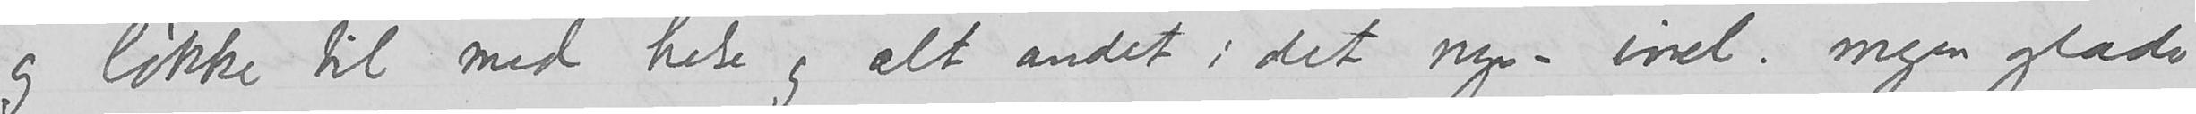og løkke til med helse og alt andet i det nye – incl. megen glæde
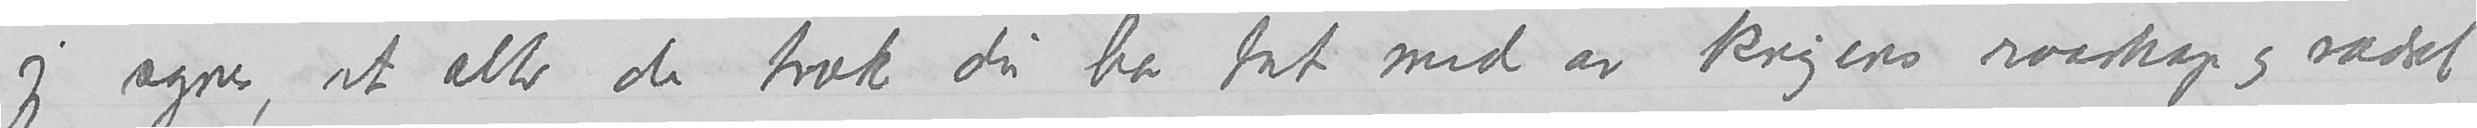jeg synes, at alle de træk du har tat med av krigens raaskap og rædsel
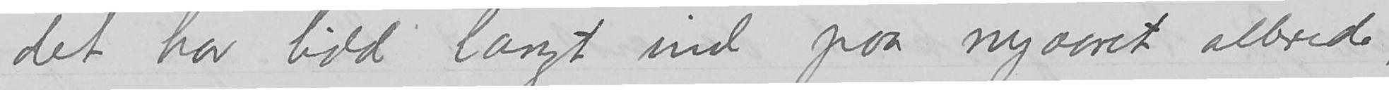det har lidd langt ind paa nyaaret allerede,
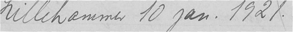Lillehammer 10. jan. 1921.
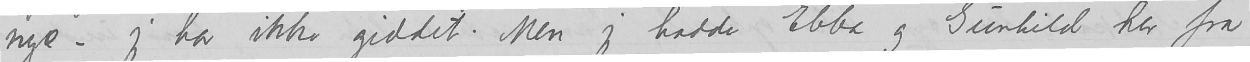nye – jeg har ikke giddet.  Men jeg hadde Ebbe og Gunhild her fra
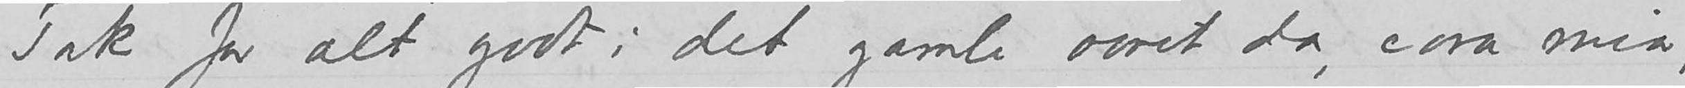Tak for alt godt i det gamle aaret da, cora mia,
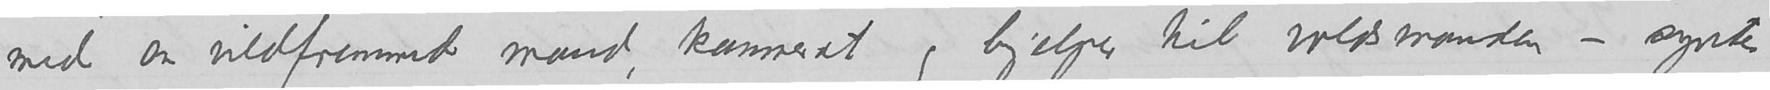med en vildfremmed mand, kammerat og hjelper til voldsmanden – syntes
